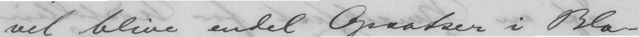vel blive endel Opsatser i Bla-
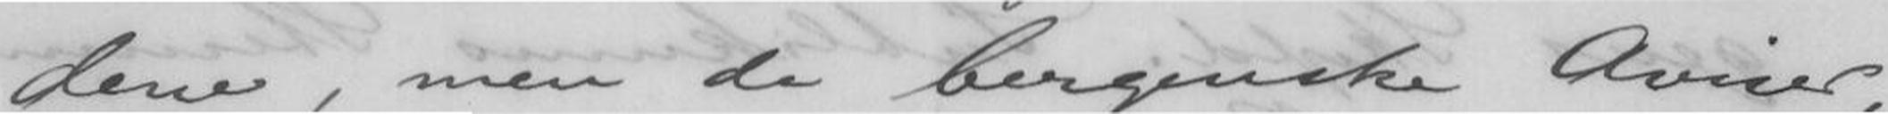dene, men de bergenske Aviser,
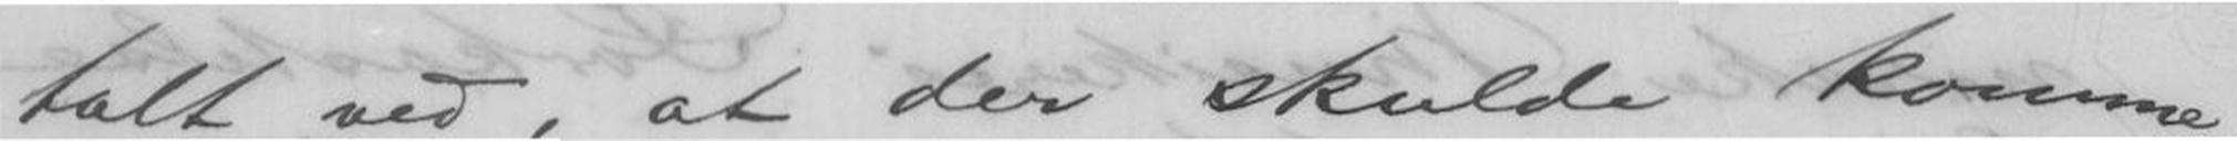talt ved, at der skulde komme
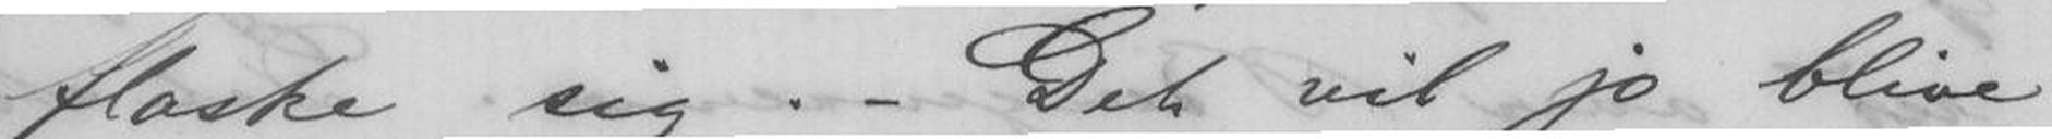flaske sig. - Det vil jo blive
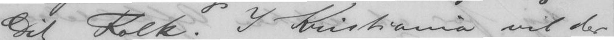Dit Folk. I Kristiania vil der
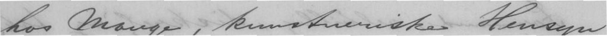hos Mange, kunstneriske Hensyn
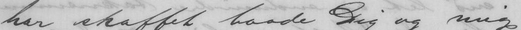har skaffet baade Dig og mig
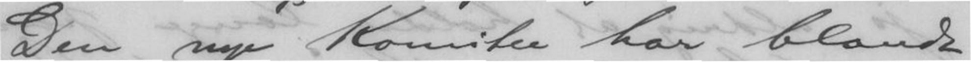Den nye Komitee har blandt
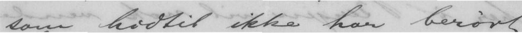som hidtil ikke har berørt
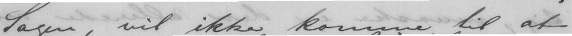Sagen, vil ikke komme til at
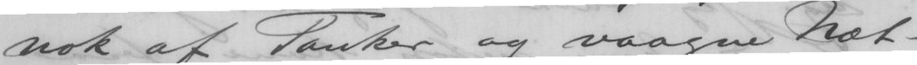nok af Tanker og vaagne Næt-
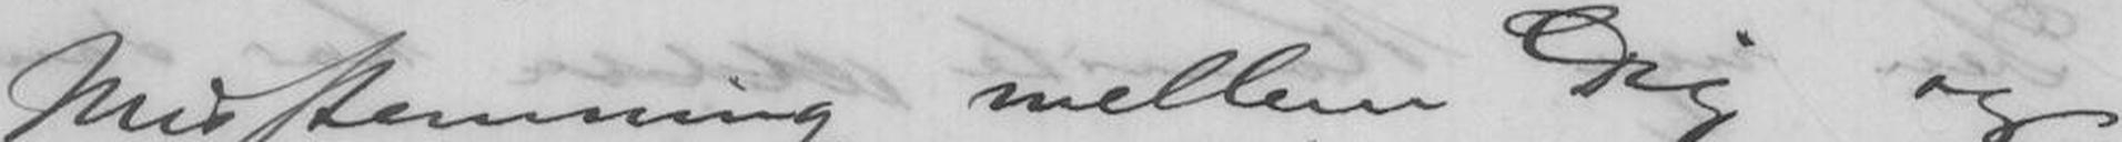Misstemning mellem Dig og
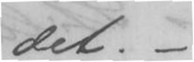det. -
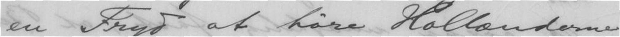en Fryd at høre Hollænderne,
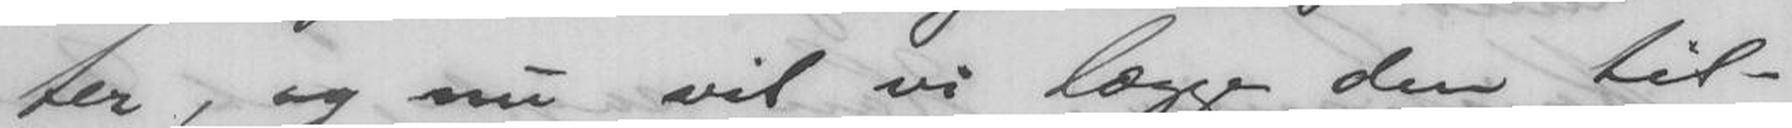ter, og nu vil vi lægge den til-
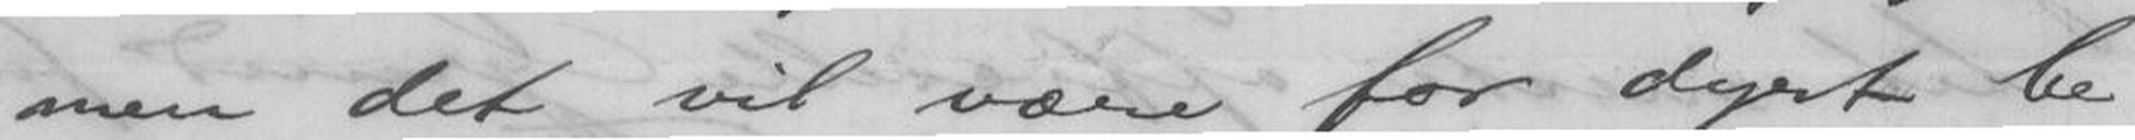men det vil være for dyrt be-
